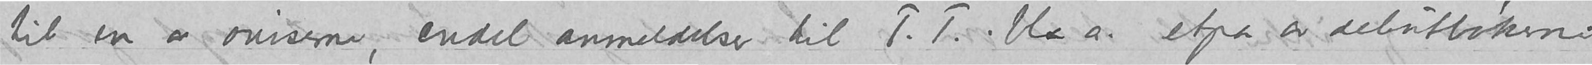til en av aviserne, en del anmeldelser til T.T. bl.a. etpar av debutbøkerne.
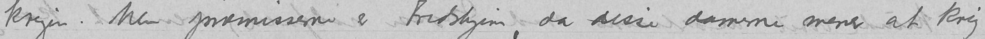krigen. Men præmisserne er Fredshjem, da disse damerne mener at krig
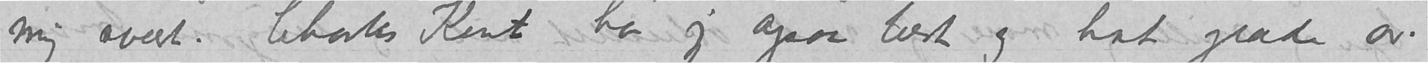mig svært. Charles Kent har jeg ogsaa læst og hat glæde av.
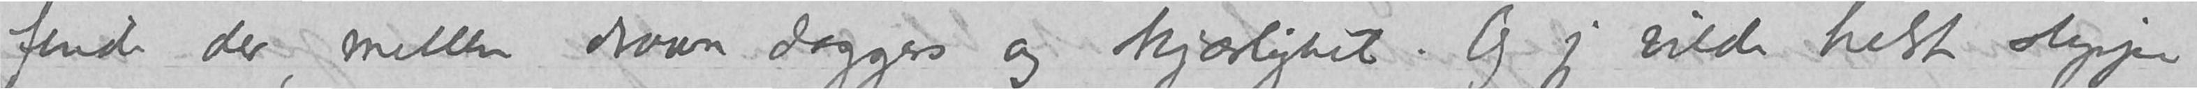finde der, mellem drawn daggers og kjærlighet. Og jeg vilde helst slippe
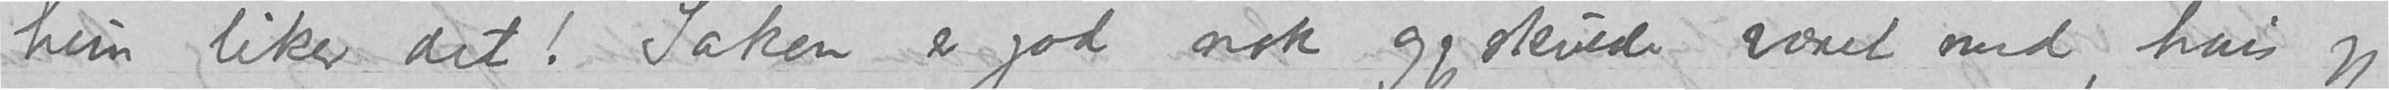hun liker det! Saken er god nok og jeg skulde været med, hvis jeg
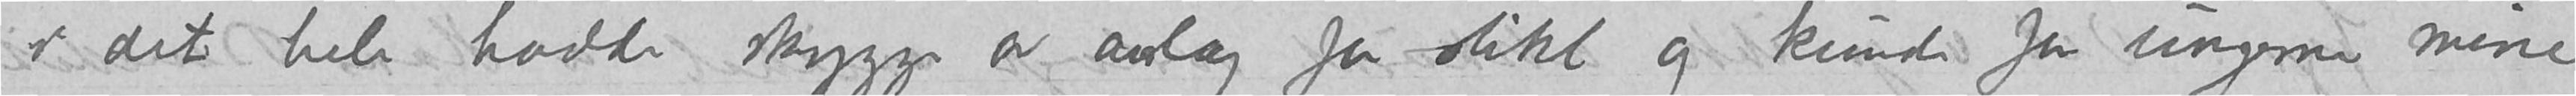i det hele hadde skygge av anlæg for slikt og kunde for ungerne mine
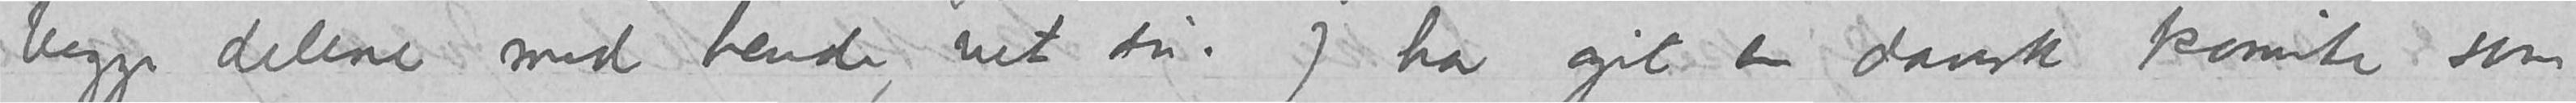begge delene med hende, vet du. Jeg har git en dansk komite som
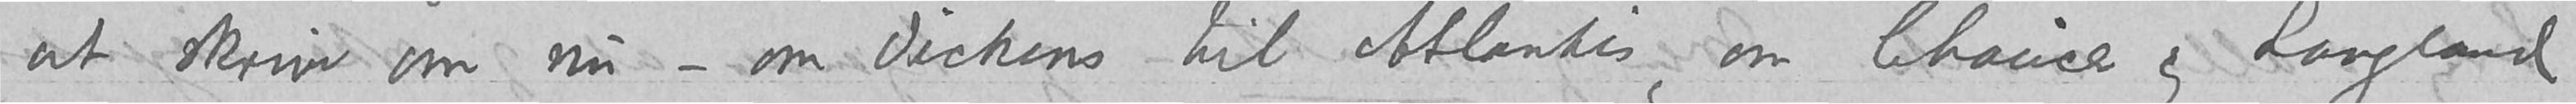at skrive om nu – om Dickens til Atlantis, om Chaucer og Langeland
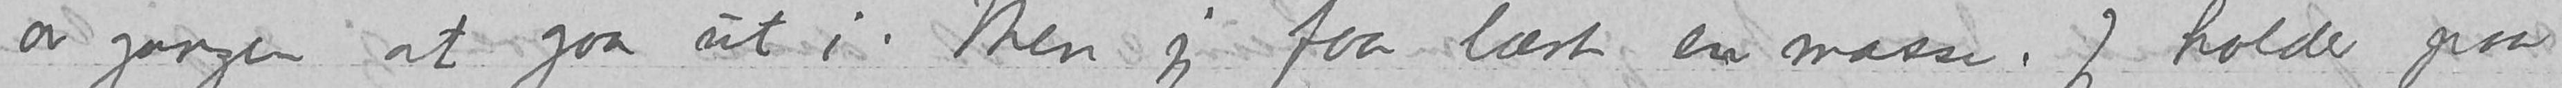av gangen at gaa ut i. Men jeg faar lært en masse. Jeg holder paa
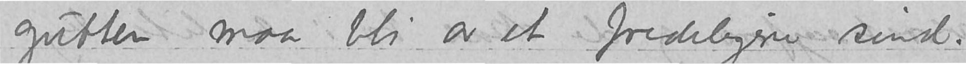gutten maa bli av et fredeligere sind.
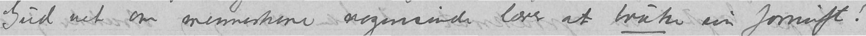Gud vet om menneskene nogensinde lærer at bruke sin fornuft!
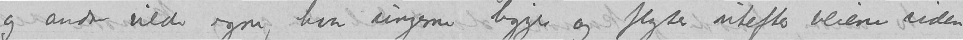og andre vilde egne, hvor ungerne ligger og flygter utefter veiene siden
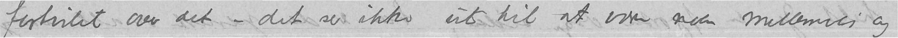fortvilet over det – det ser ikke ut til at være noen mellemvei og
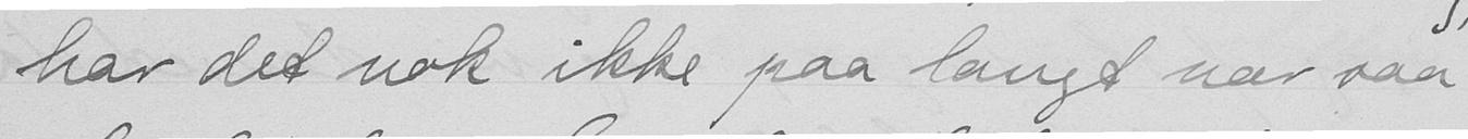har det nok ikke paa langt nær saa
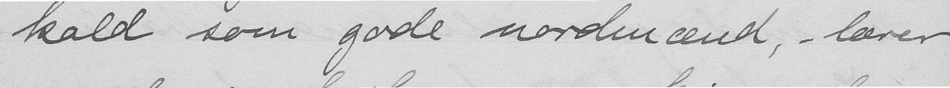fald som gode nordmænd, - lærer
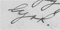lyst.
